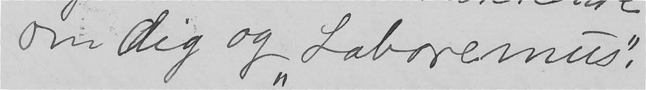om dig og "Laboremus".
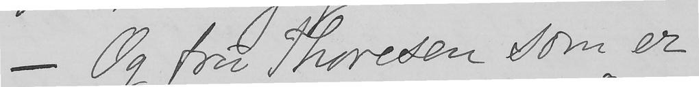- Og fru Thoresen som er
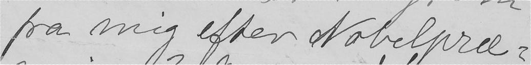fra mig efter Nobelpræ-
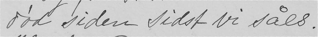død siden sidst vi såes.
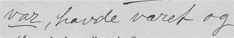var, havde været og
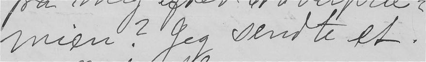mien? Jeg sendte et.
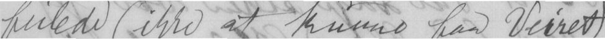feilede (ikke at kunne faa Veiret)
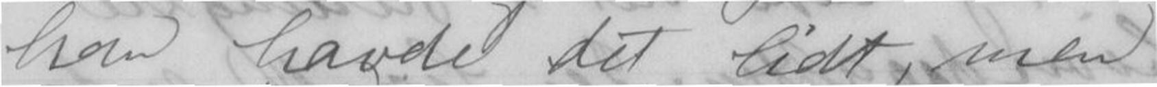han havde det lidt, men
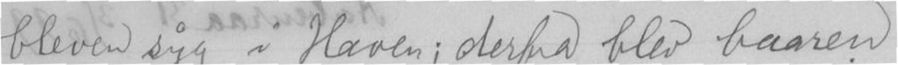bleven syg i Haven; derfra blev baaren
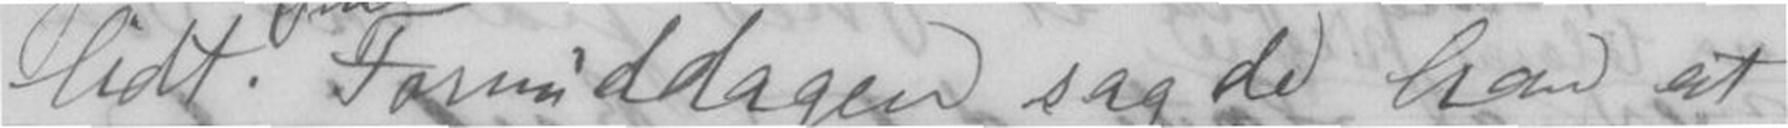lidt. Formiddagen sagde han at
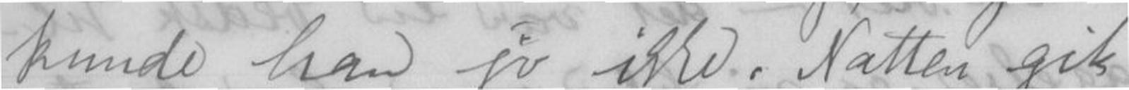kunde han jo ikke. Natten gik
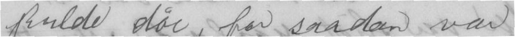skulde dø, for saadan var
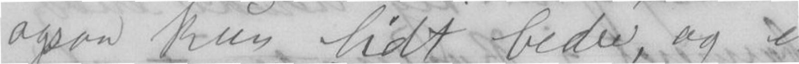ogsaa hun lidt bedre, og et
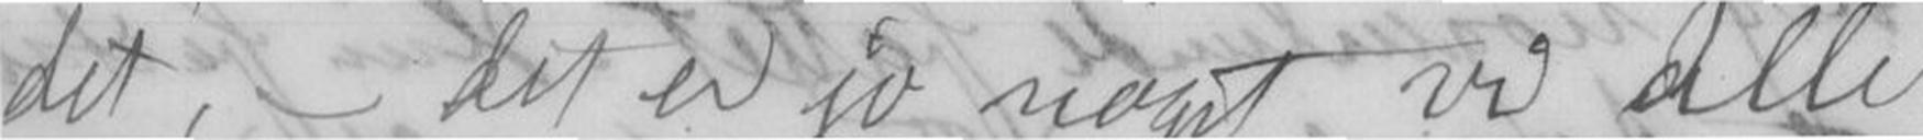det, - det er jo noget vi alle
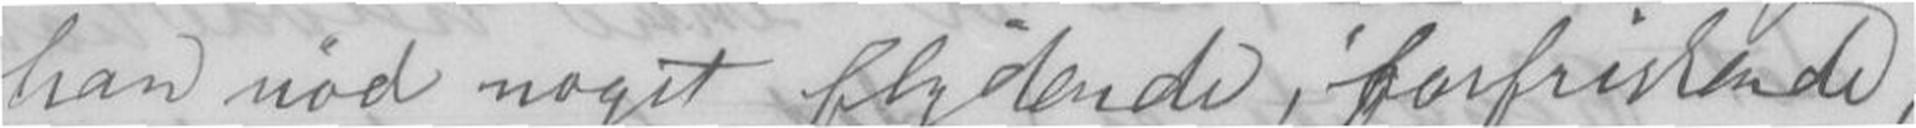han nød noget flydende, forfriskende,
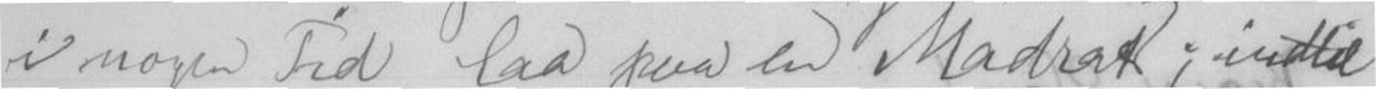i nogen Tid laa paa en Madras, indtil
